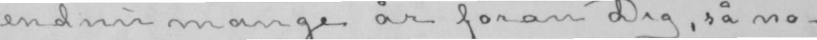endnu mange år foran Dig, så no-
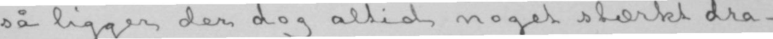så ligger der dog altid noget stærkt dra-
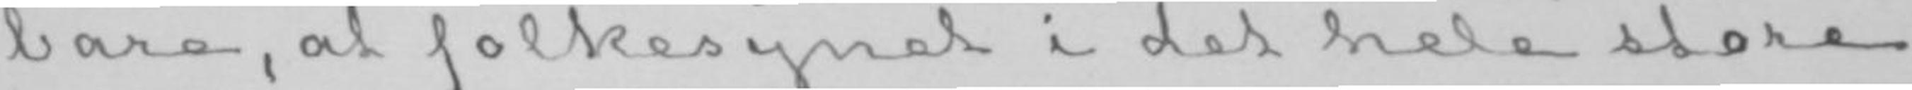bare, at folkesynet i det hele store
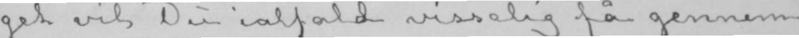get vil Du ialfald visselig få gennem-
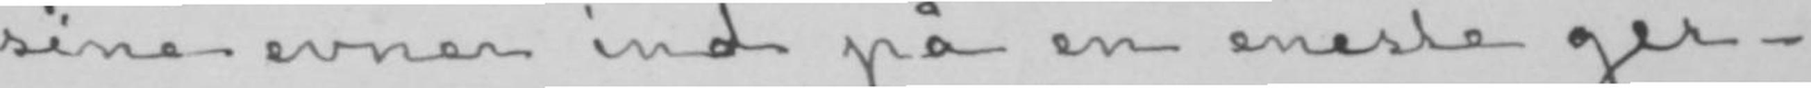sine evner ind på en eneste ger-
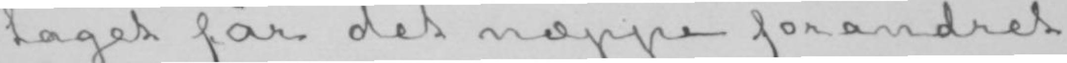taget får det næppe forandret
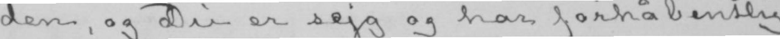den, og Du er sejg og har forhåbentlig
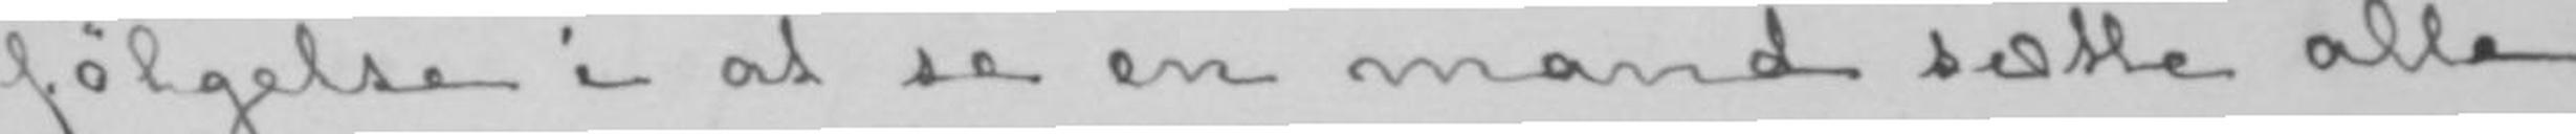følgelse i at se en mand sætte alle
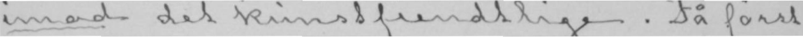imod det kunstfiendtlige. Få først
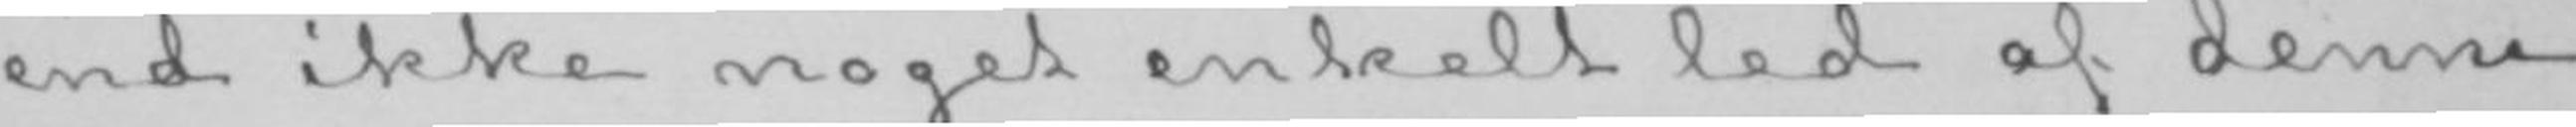end ikke noget enkelt led af denne
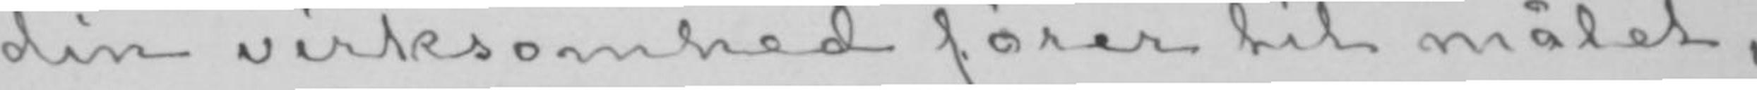din virksomhed fører til målet,
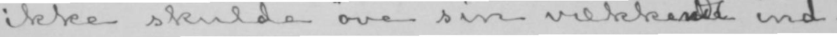ikke skulde øve sin vækkende ind-
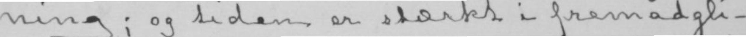ning; og tiden er stærkt i fremadgli-
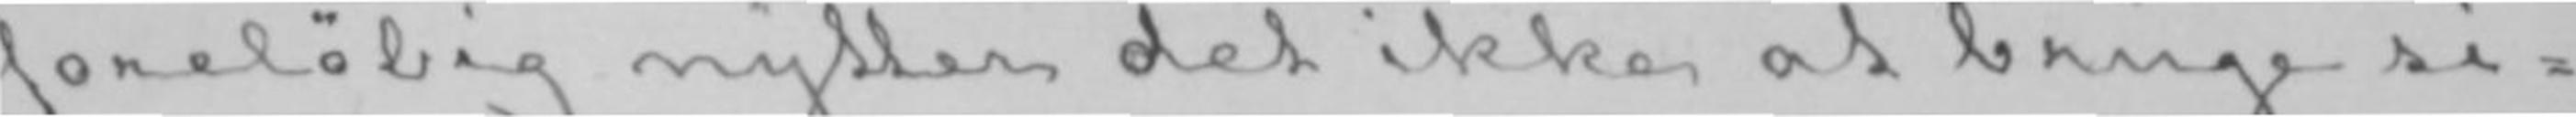foreløbig nytter det ikke at bruge si-
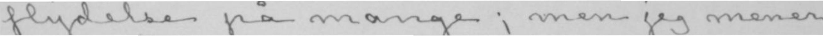flydelse på mange; men jeg mener
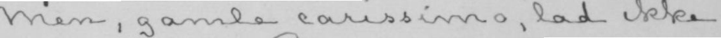Men, gamle carissimo, lad ikke
